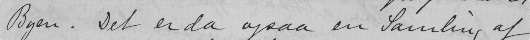Byen. Det er da ogsaa en Samling af
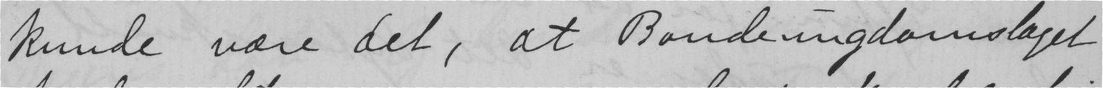kunde være det, at Bondeungdomslaget
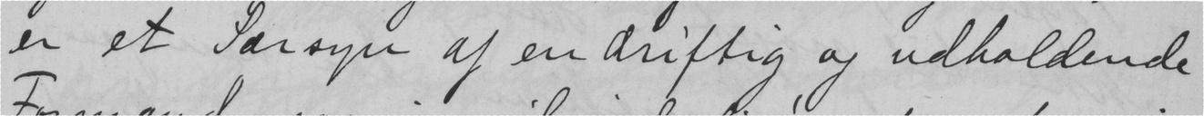er et Særsyn af en driftig og udholdende
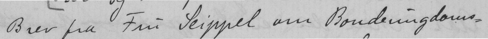Brev fra Fru Seippel om Bondeungdoms-
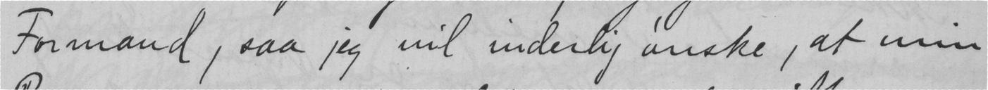Formand, saa jeg vil inderlig ønske, at min
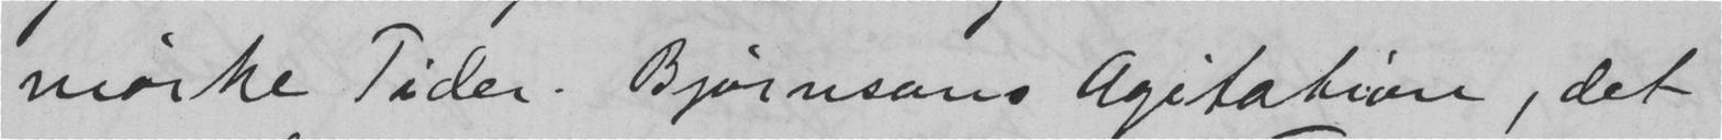mørke Tider. Bjørnsons Agitation, det
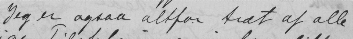Jeg er ogsaa altfor træt af alle
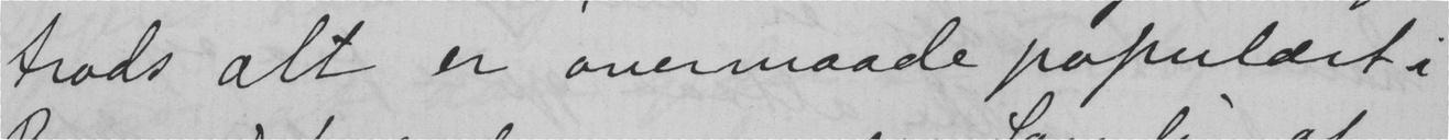trods alt er overmaade populært i
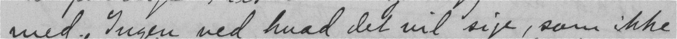med. Ingen ved hvad det vil sige, som ikke
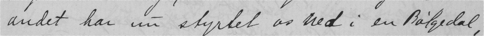andet har nu styrtet os ned i en Bølgedal,
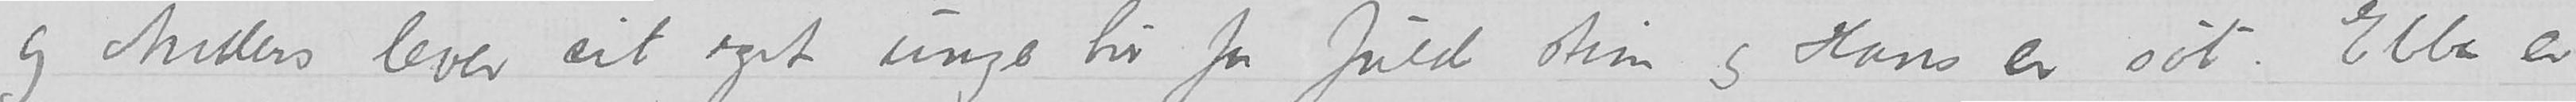og Anders lever sit eget unge liv for fuld stim og Hans er søt. Ebba er
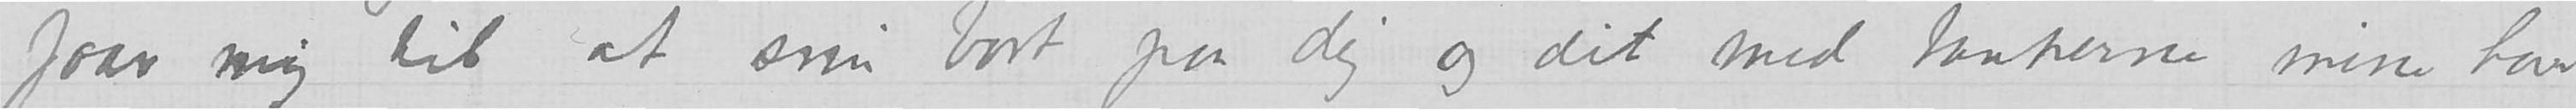faar mig til at snu bort paa dig og dit med tankerne mine har
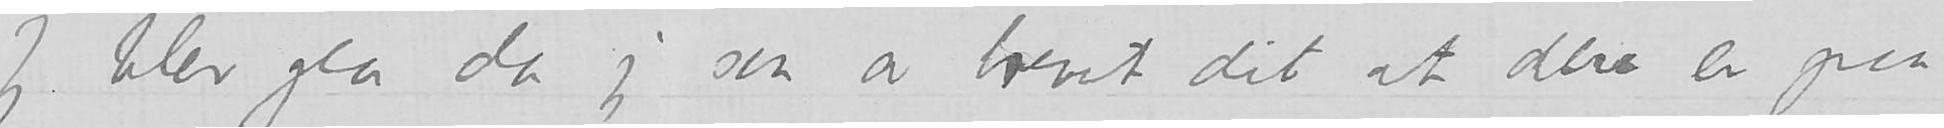Jeg blev gla da jeg saa av brevet dit at dere er paa
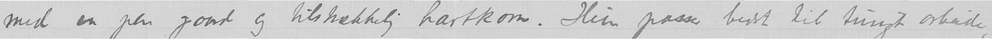med en pen gaard og tilstrækkelig hartkorn. Hun passer bedst til tungt arbeide,
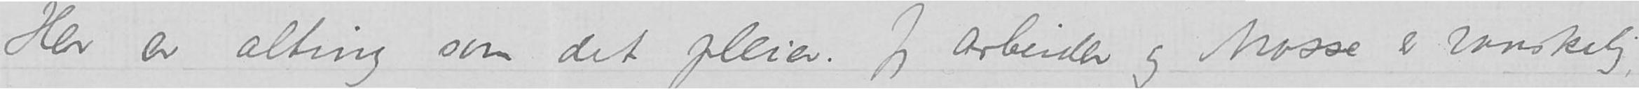Her er alting som det pleier. Jeg arbeider og Mosse er vanskelig,
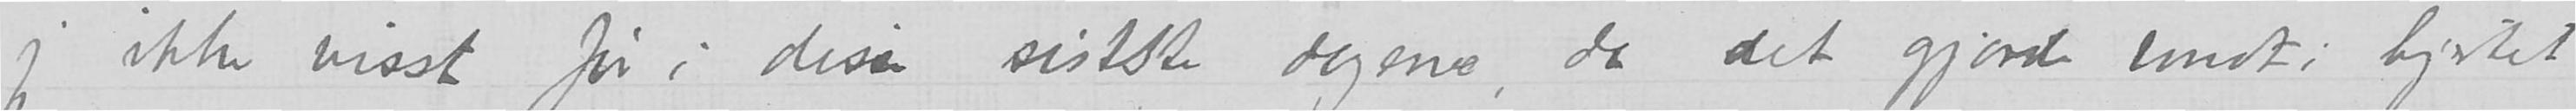jeg ikke visst før i disse sidste dagene,  og det gjorde vondt i hjertet
